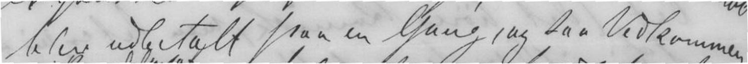blev udbetalt paa en Gang, og saa Vedkommen-
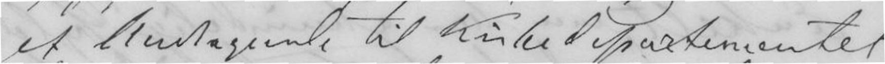et Andragende til Kirkedepartementet
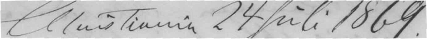Christiania 24 Juli 1869.
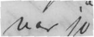var jo
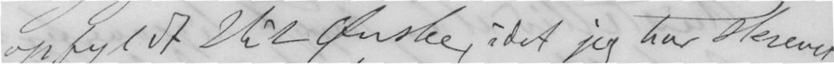opfyldt Dit Ønske, idet jeg har skrevet
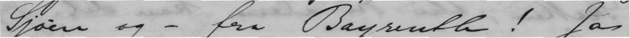Sjøen og - fra Bayreuth! Ja,
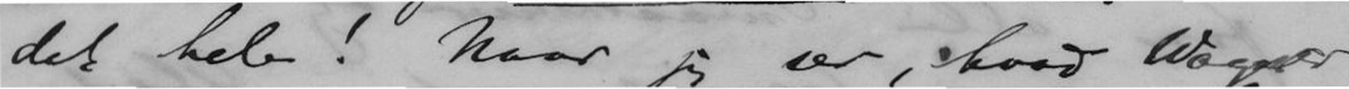det hele! Naar jeg ser, hvad Wagner
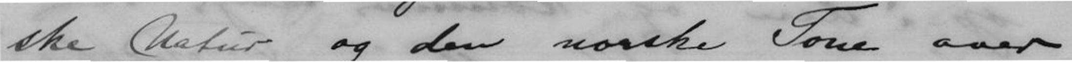ske Natur og den norske Tone over
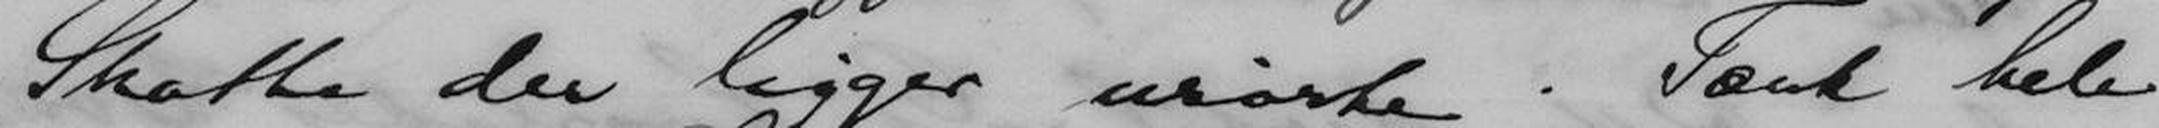Skatte der ligger urørte. Tænk hele
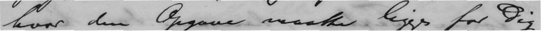hvor den Opgave maatte ligge for Dig.
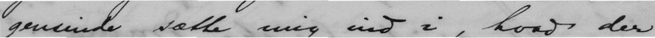gensinde sætte mig ind i, hvad der
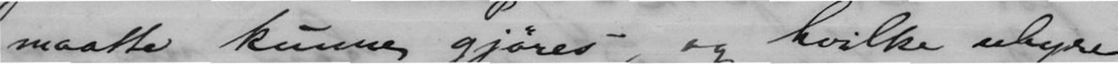maatte kunne gjøres, og hvilke uhyre
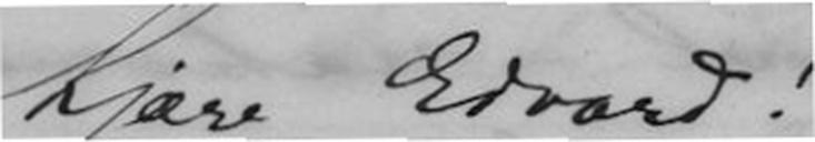Kjære Edvard!
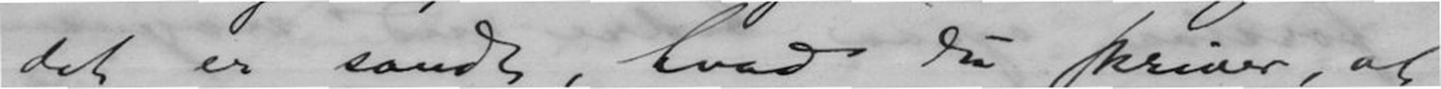det er sandt, hvad du skriver, at
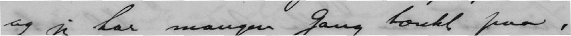og jeg har mangen Gang tænkt paa,
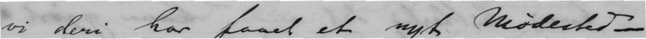vi deri har faaet et nyt Mødested -
